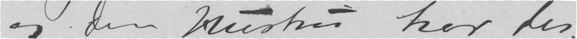og din Hustru bor des-
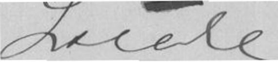Locale
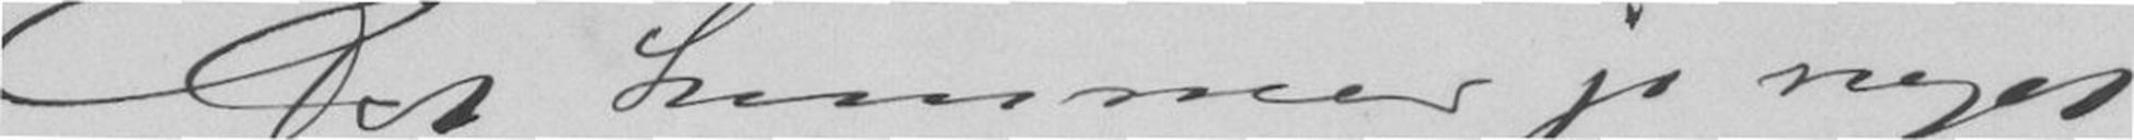Det kommer jo noget
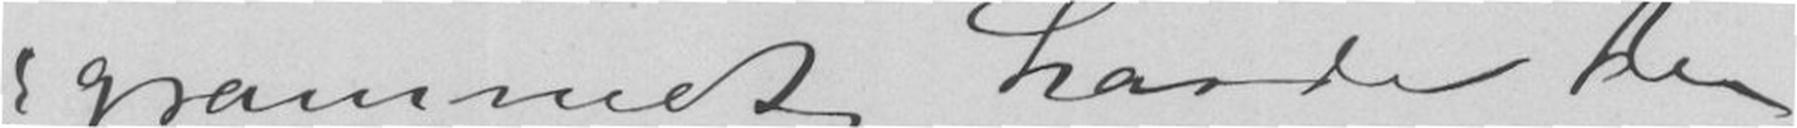grammet havde da
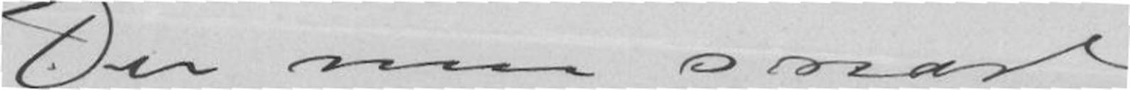Du maa snart
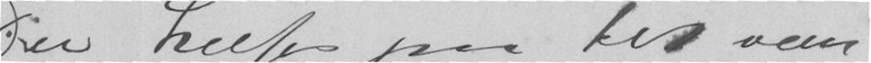Du hilses paa det ven
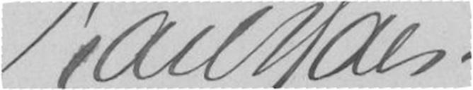Karl Hals.
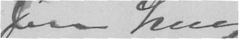Din heng.
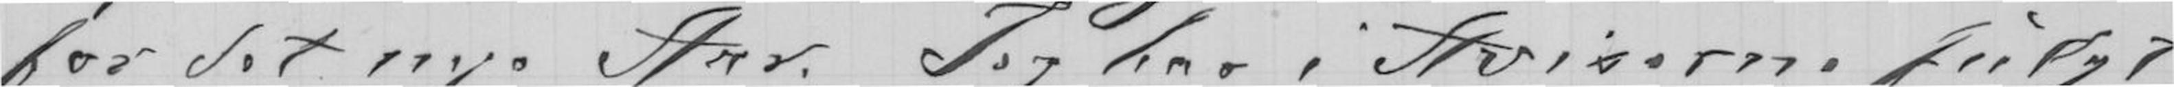for det nye Aar. Jeg har i Aviserne fulgt
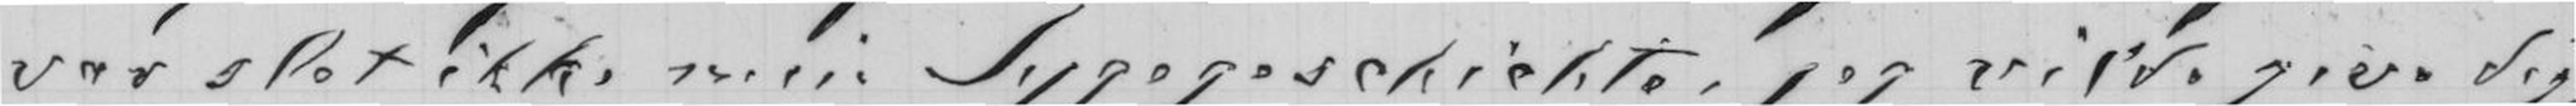var slet ikke min Sygegeschichte, jeg vilde give dig,
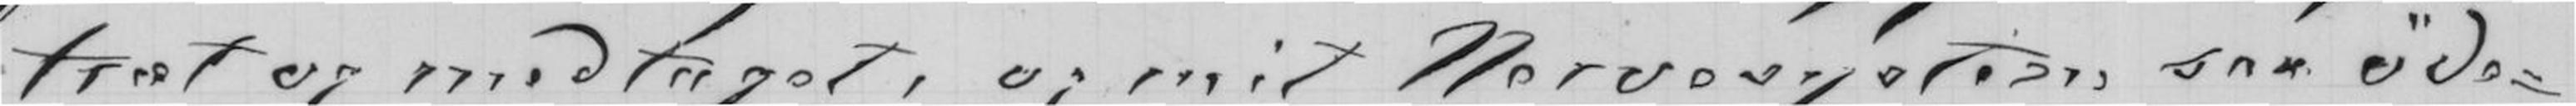træt og medtaget, og mit Nervesystem saa øde-
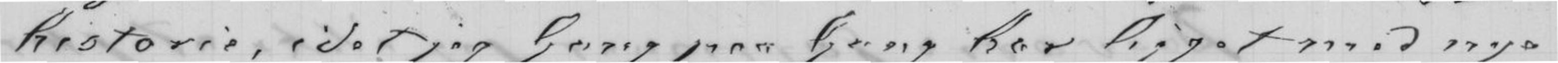historie, idet jeg Gang paa Gang har ligget med nye
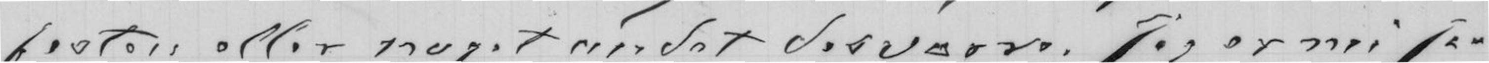festen eller noget andet desværre. Jeg er nu saa
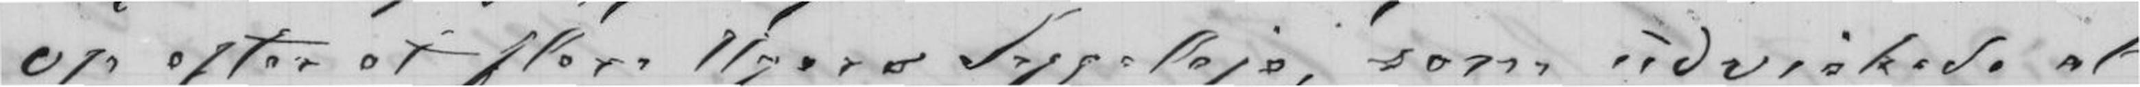op efter et flere Ugers Sygeleje, som udviskede al
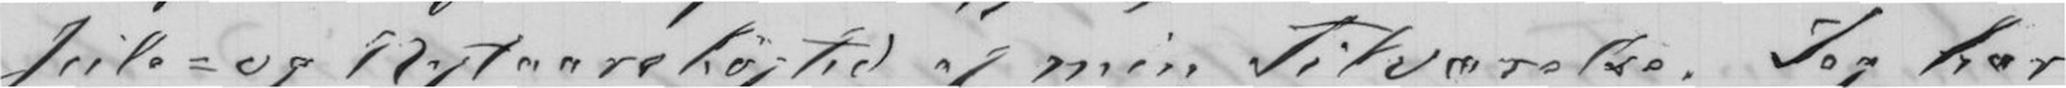Jule- og Nytaarshøjtid af min Tilværelse. Jeg har
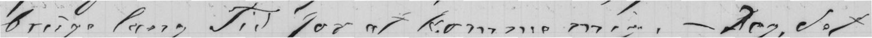bruge lang Tid for at komme mig. - Dog, det
